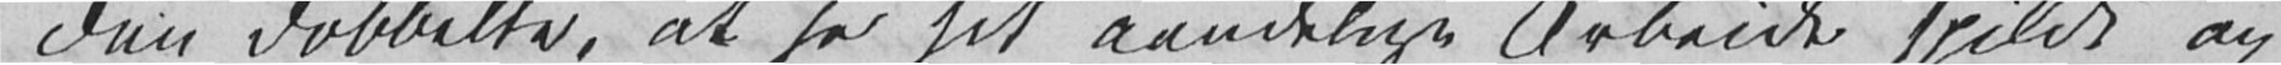den dobbelte, at se sit aandelige Arbeide spildt og
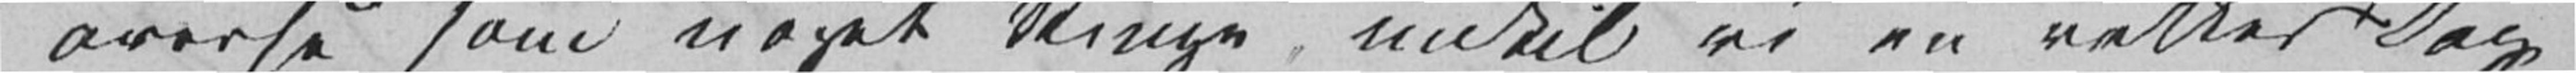overse som noget Ringe, indtil vi en vakker Dag
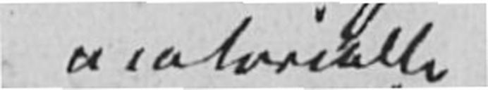materielle
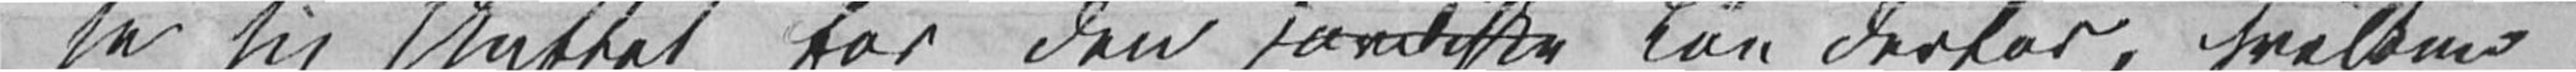se sig skuffet for den jordiske Løn derfor, hvilken
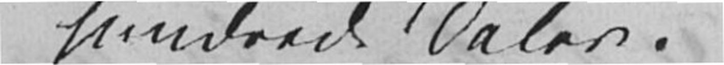hundrede Daler.
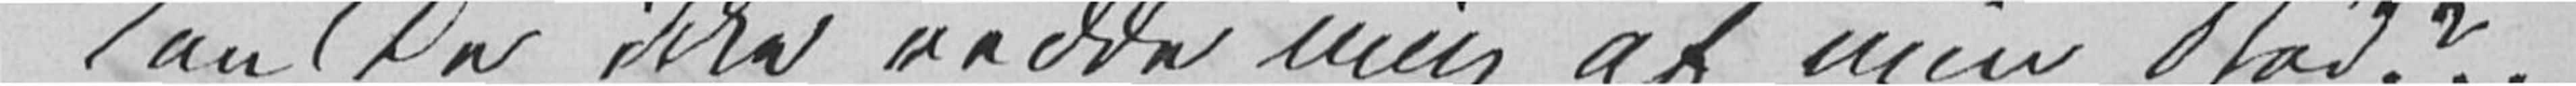Kan De ikke redde mig af min Nød?..
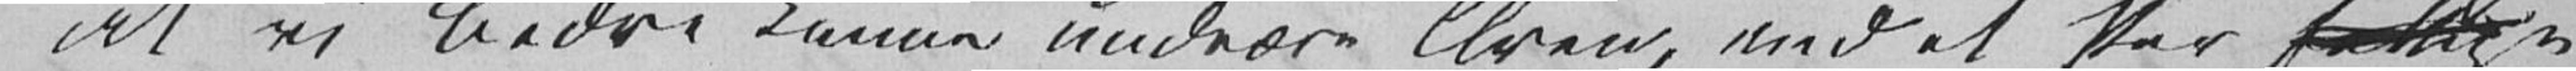at vi bedre kunne undvære Æren, end et Par fattige
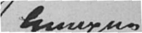lumpne
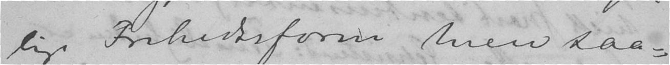lige Frihedsform, men saa-
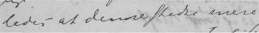ledes at denne stedse mere
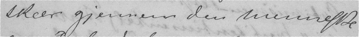skeer gjennem den menneske-
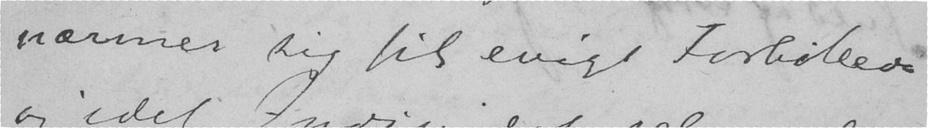nærmer sig sit evige Forbillede
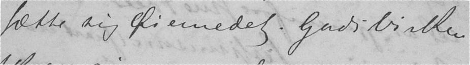sætte sig Øiemedet. Guds Virken
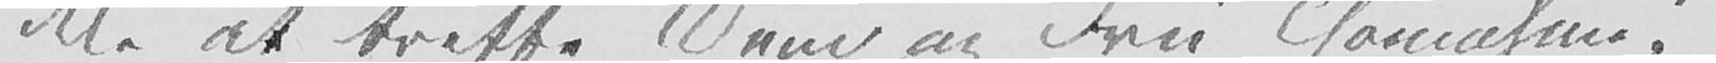ikke at treffe Dem og Fru Thomasine!
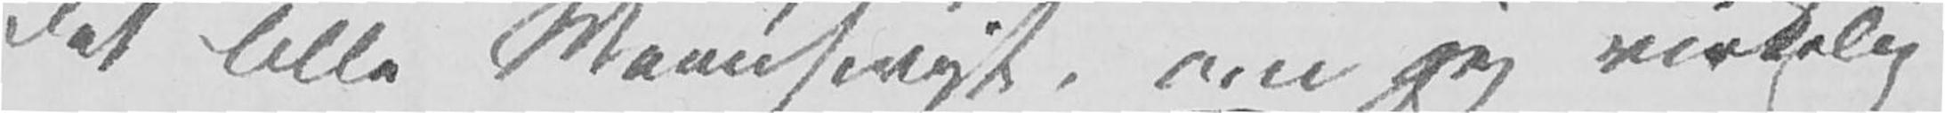det lille Manuscript, om jeg virkelig
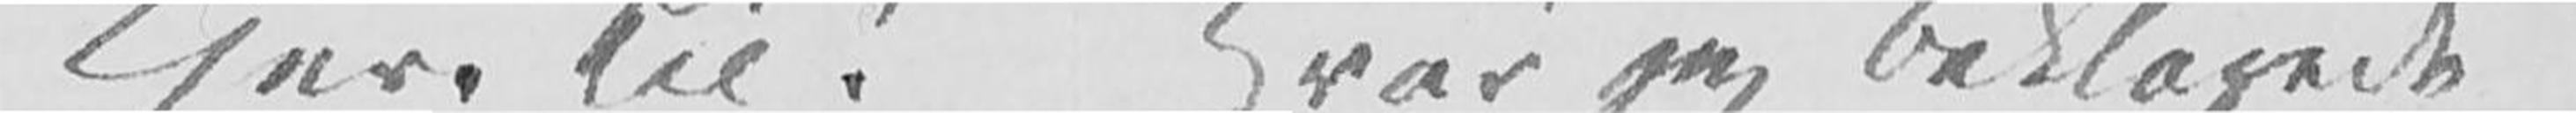Kjere Lie! Hvor jeg beklagede
